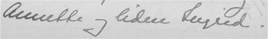Annette og liden Ingrid.
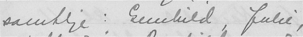samtlige: Gunhild, Julie,
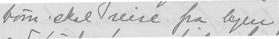børn skal reise fra byen
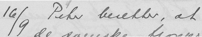16/9 Peter beretter, at
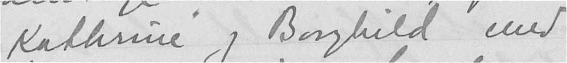Kathrine og Borghild med
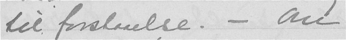til forstaaelse. - Om
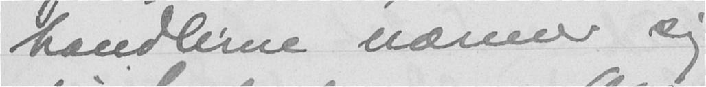handlerne nærmer sig
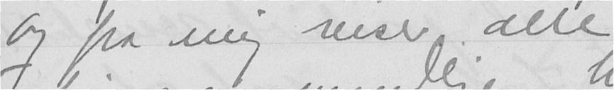Og fra mig reiser alle
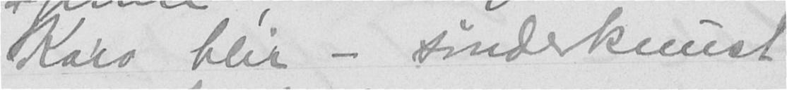Karo blir - sønderknust
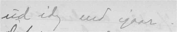ud idag end gaar.
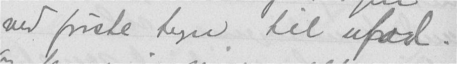ved første tegn til ufred.
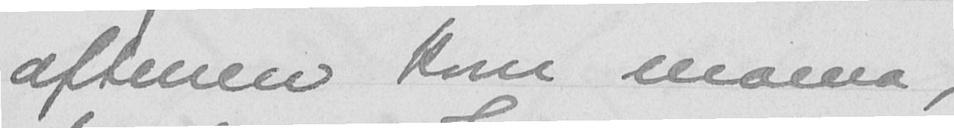aftenen kom mama,
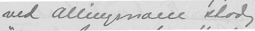ved Allingsvalle stadig
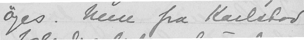øjes. Men fra Karlstad
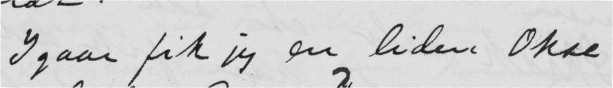Igaar fik jeg en liden Okse
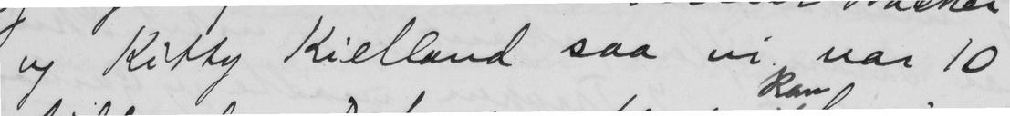og Kitty Kielland saa vi var 10
